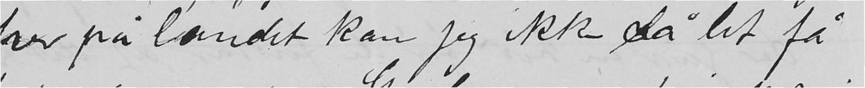her på landet kan jeg ikke så let få
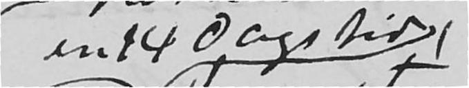en 14 dags tid,
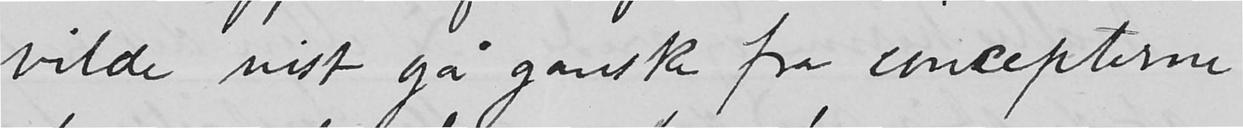vilde vist gå ganske fra concepterne
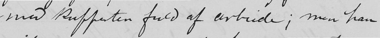med kufferten fuld af arbeide; men han
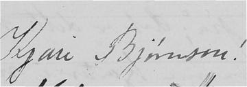Kjære Bjørnson!
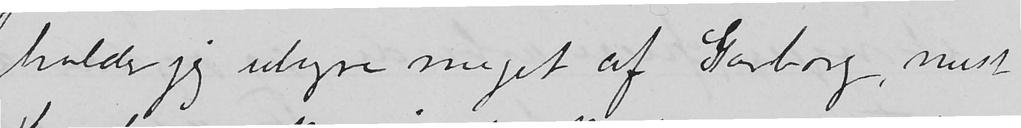holder jeg uhyre meget af Garborg, mest
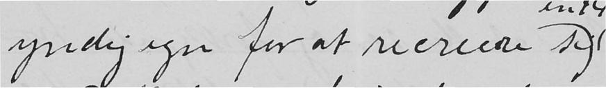yndig egn for at recreere sig
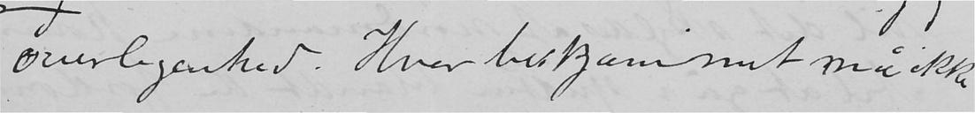overlegenhed. Hvor beskjemmet må ikke
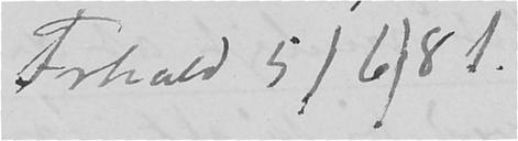Fr.hald 5/6/81.
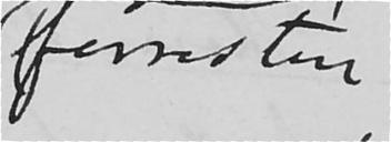forresten
